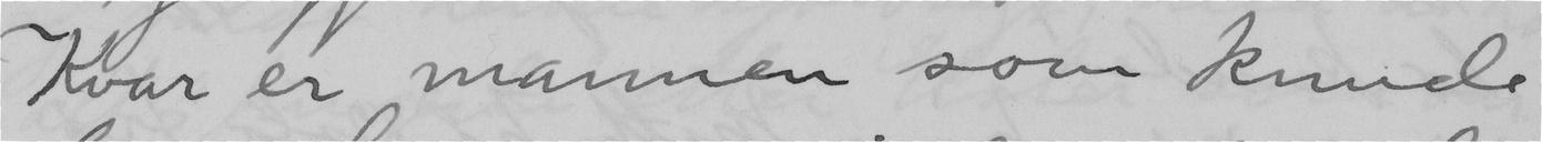Kvar er mannen som kunde
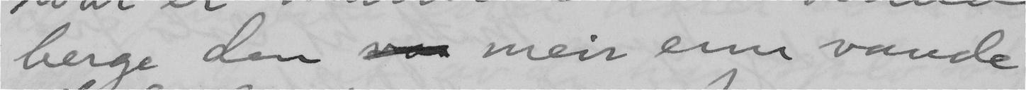berge den  meir enn vonde
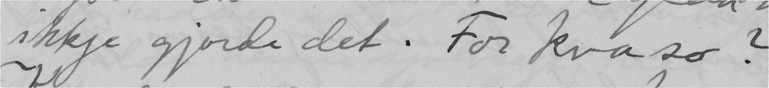ikkje gjorde det. For kva so?
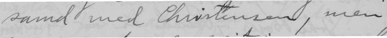samd med Christensen, men
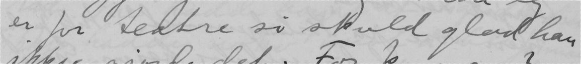er for teatre si skuld glad han
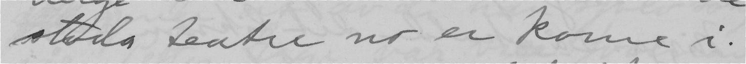stoda teatre no er kome i.
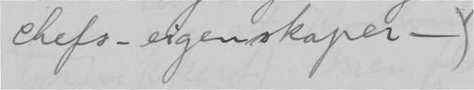Chefs-eigenskaper –
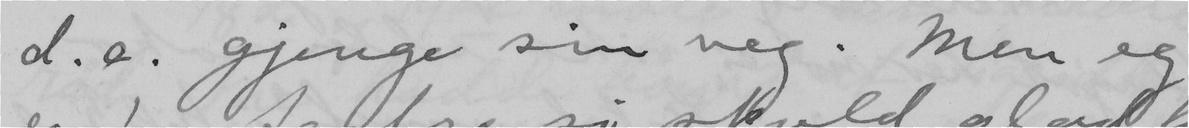d.e. gjenge sin veg. Men eg
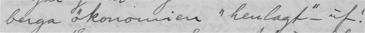berga ökonomien "henlagt" – ut!
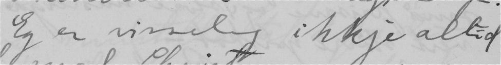Eg er visselig ikkje altid
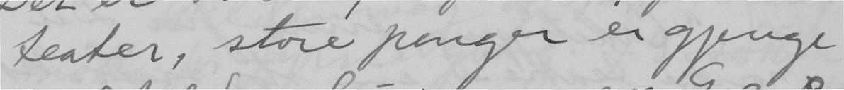teater, store penger er gjenge
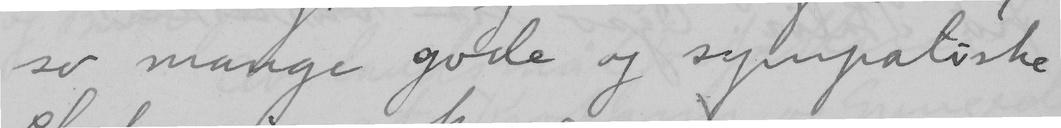so mange gode og sympatiske
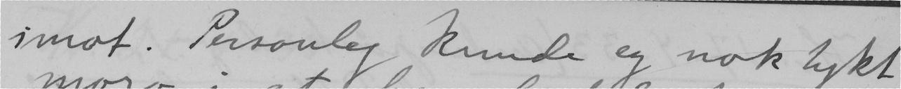imot. Personleg kunde eg nok lykt
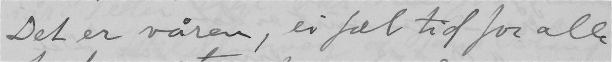Det er våren, ei fæl tid for alle
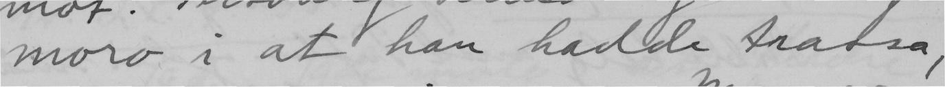moro i at han hadde tratsa
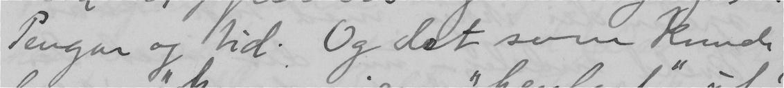Pengar og tid. Og det som kunde
